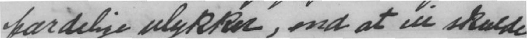færdelige ulykker, end at vi skulde
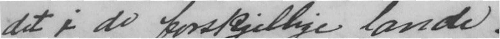det i de forskjellige lande.
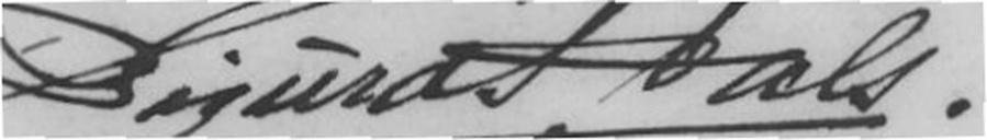Sigurd Hals.
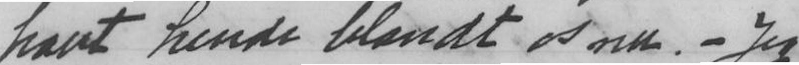havt hende blandt os nu. - Jeg
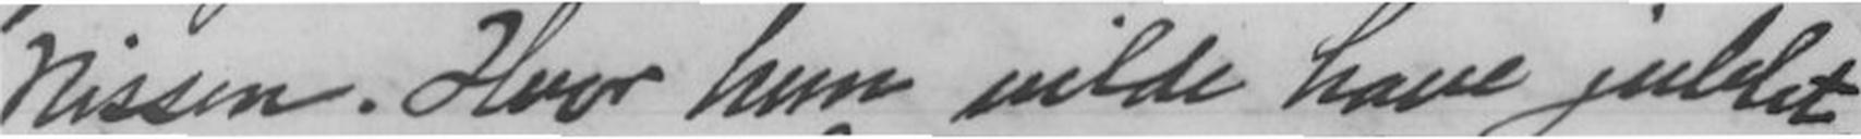Nissen. Hvor hun vilde have jublet,
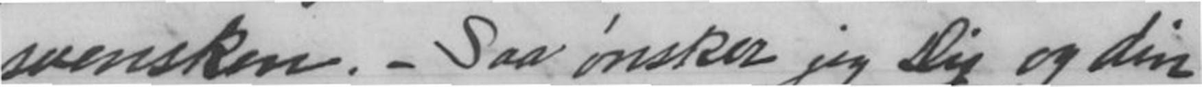svensken. - Saa ønsker jeg Dig og din
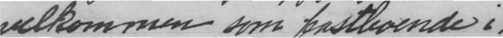velkommen som fastboende i
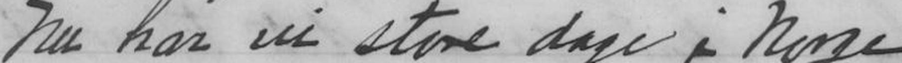Nu har vi store dage i Norge,
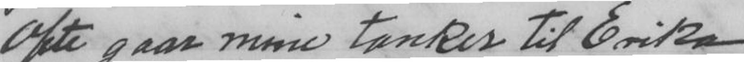Ofte gaar mine tanker til Erika
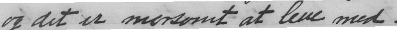og det er morsomt at leve med.
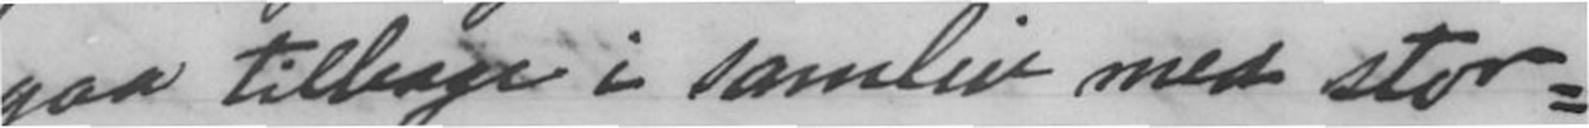gaa tilbage i samliv med stor-
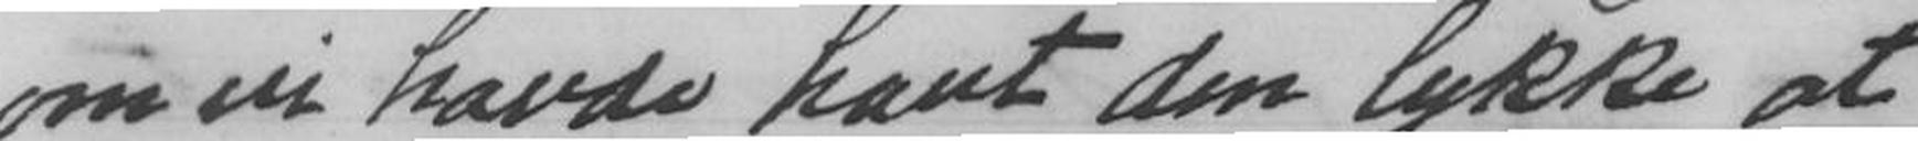om vi havde havt den lykke at
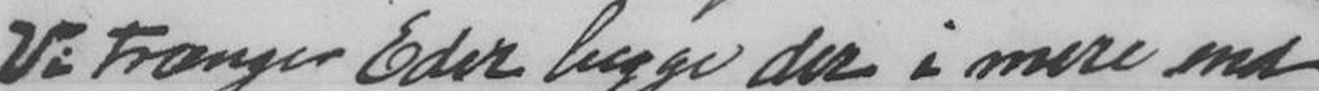Vi trænger Eder begge der i mere end
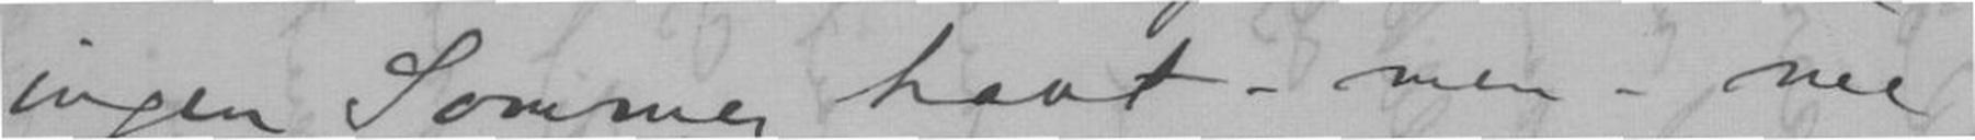ingen Sommer havt - men - nu
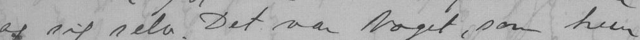og sig selv. Det var Noget, som hun
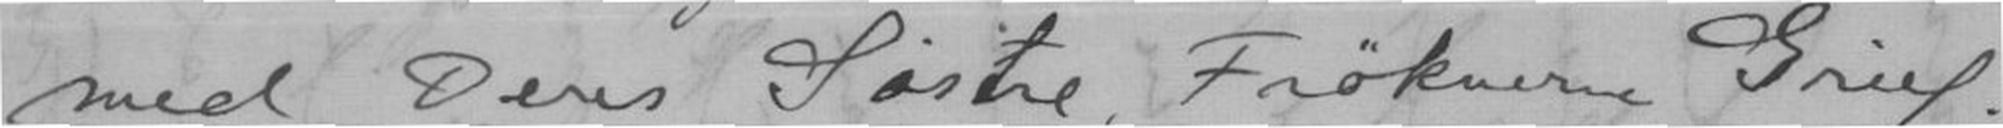med Deres Søstre, Frøknerne Grieg.
